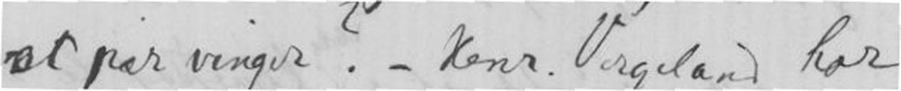et par vinger? - Henr. Vergeland har
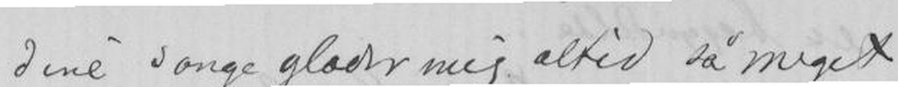dine sange glæder mig altid så meget
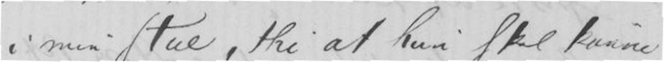i min Stue, thi at hun skal kunne
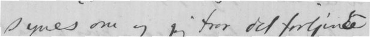synes om og jeg tror det fortjente
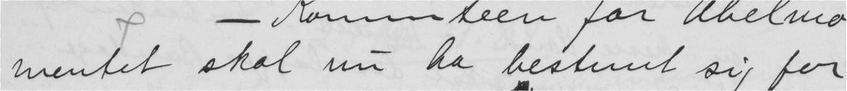mentet skal nu ha bestemt sig for
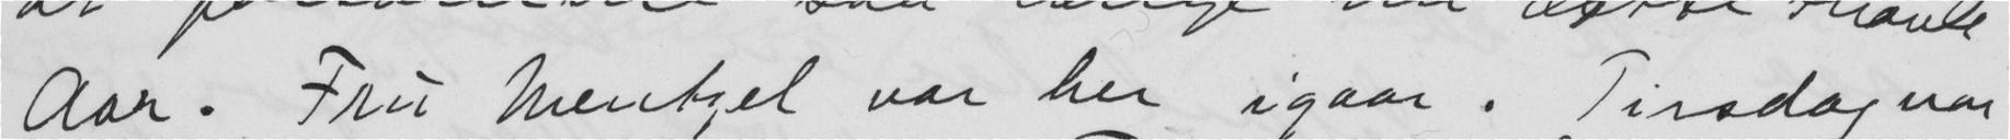Aar. Fru Wentzel var her igaar. Tirsdag var
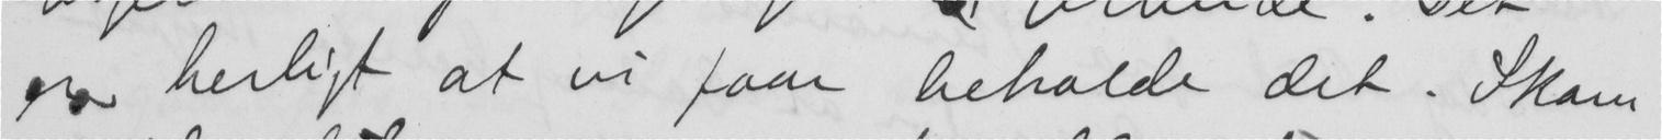en herligt at vi faar beholde det. Skam
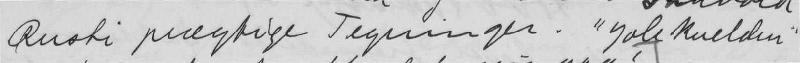Rusti prægtige Tegninger. "Jolekvelden"
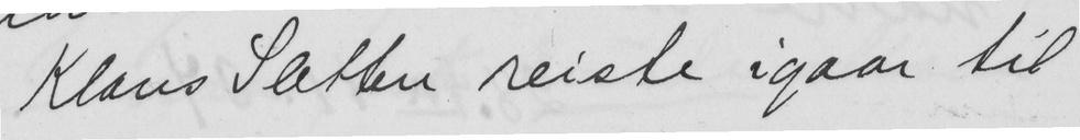Klaus Sletten reiste igaar til
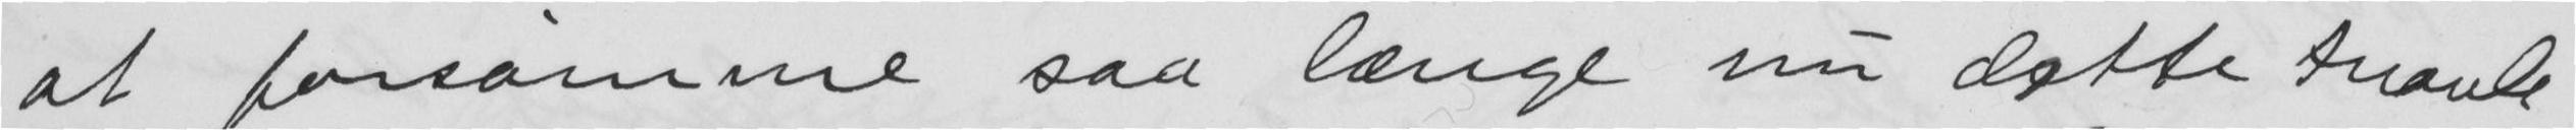at forsømme saa længe nu dette travle
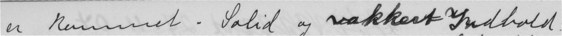er kommet. Solid og vakkert Indhold.
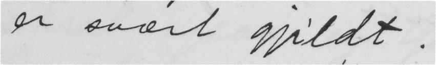er svært gjildt.
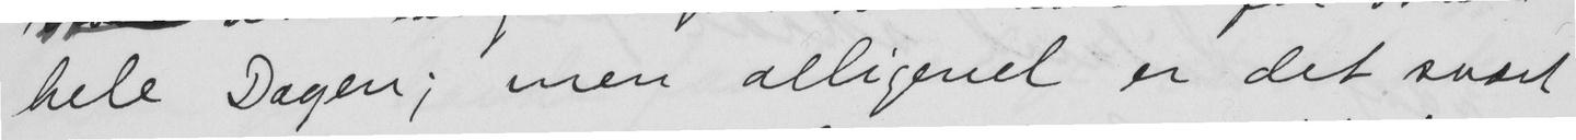hele Dagen; men alligevel er det svært
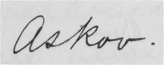Askov.
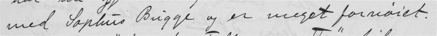med Sophus Bugge og er meget fornøiet.
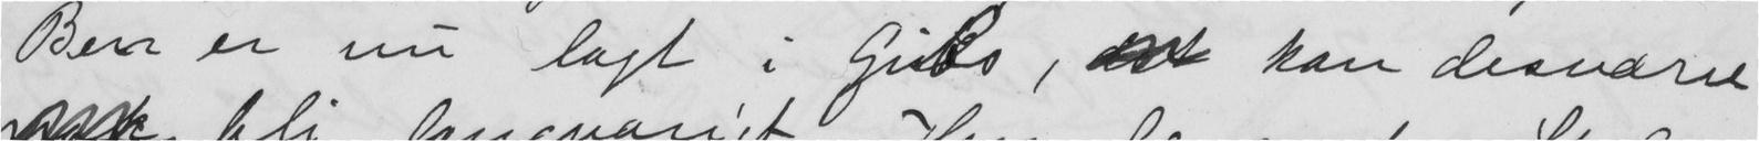Ben er nu lagt i Gibs,  kan desværre
